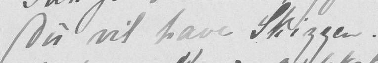Du vil have Skizzen.
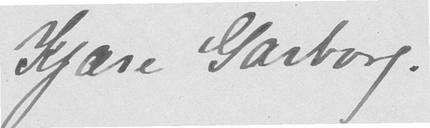Kjære Garborg.!
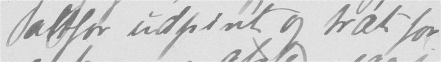altfor udpint og træt for
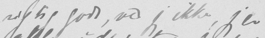rigtig godt, ved jeg ikke, jeg er
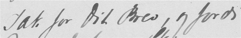Tak for dit Brev, og fordi
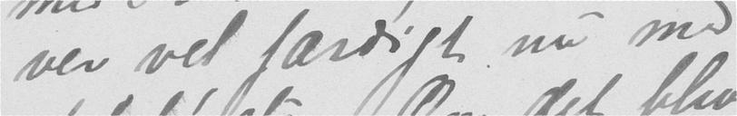ver vel færdigt nu med
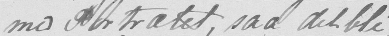med Portrætet, saa det bli-
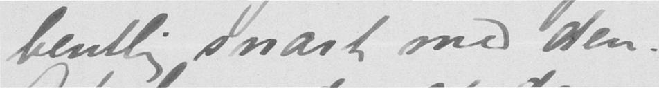bentlig snart med den.
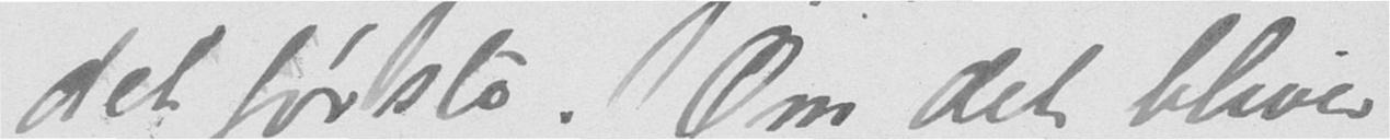det første. Om det bliver
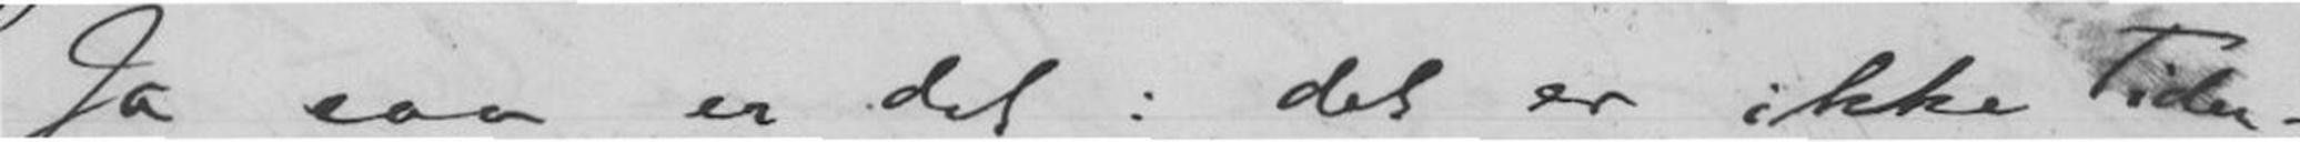Ja saa er det: det er ikke Tiden -
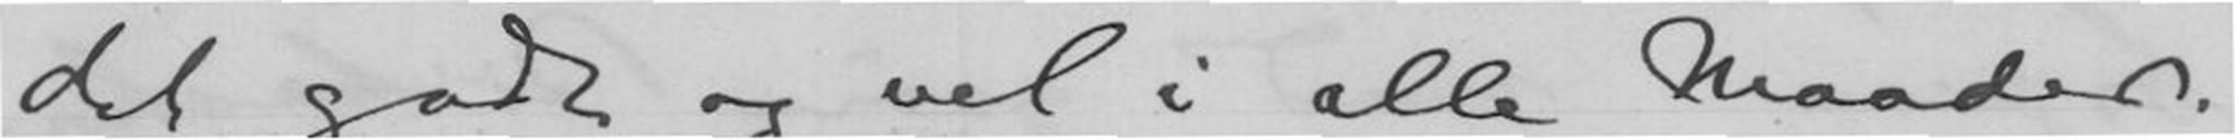det godt og vel i alle Maader.
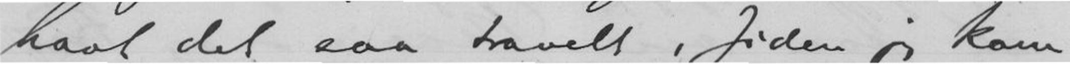havt det saa travelt, siden jeg kom
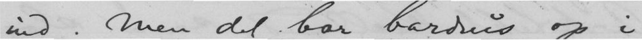ind. Men det bar bardus op i
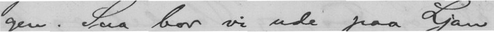gen. Saa bor vi ude paa Ljan
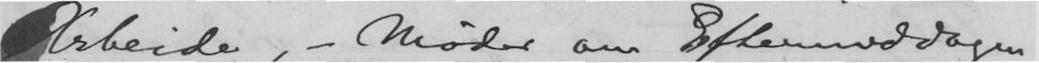Arbeide, - Møder om Eftermiddagen
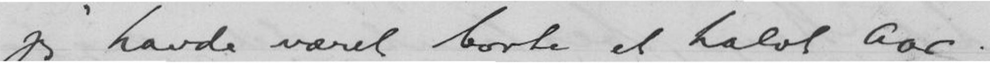jeg havde været borte et halvt Aar.
